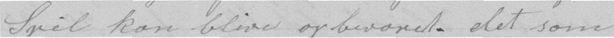Spil kan blive opbevaret. det som
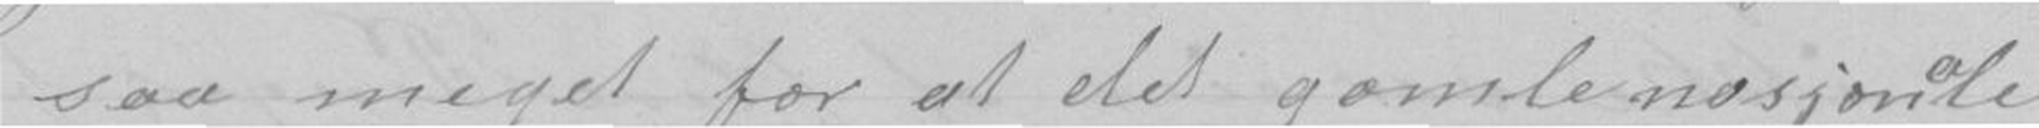saa meget for at det gamle nasjonale
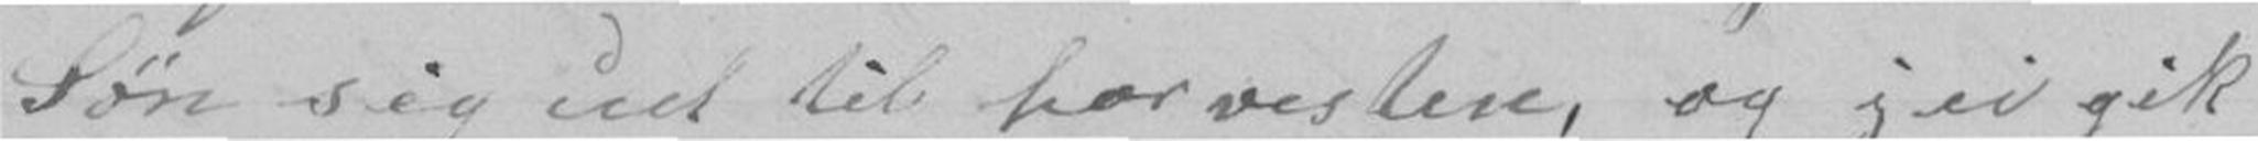Søn sig ud til harvestere, og jei gik
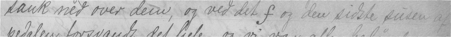sank ned over dem, og ved det f og den sidste susen af
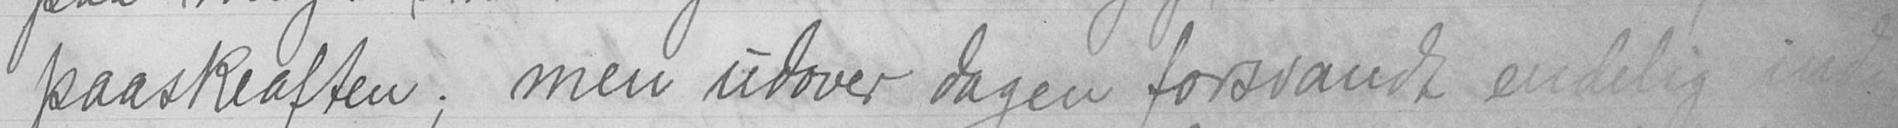paaskeaften; men udover dagen forsvandt endelig ind-
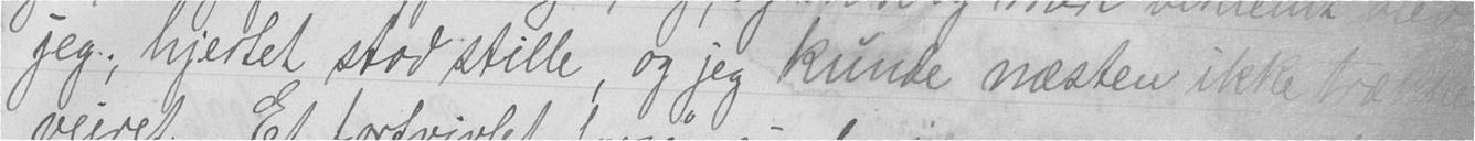jeg; hjertet stod stille, og jeg kunde næsten ikke trække
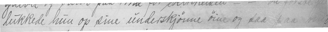lukkede hun op sine underskjønne øine og saa paa mig
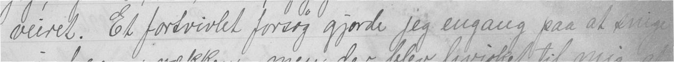veiret. Et fortvivlet forsøg gjorde jeg engang paa at snige
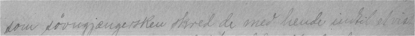som søvngjængersken skred de med hende indtil et vist
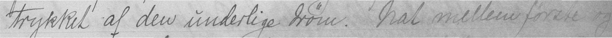trykket af den underlige drøm. Nat mellem første og
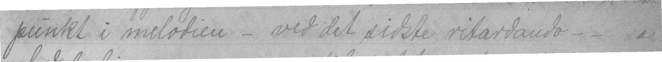punkt i melodien - ved det sidste ritardando - - saa
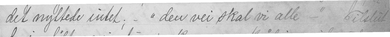det nyttede intet; - "den vei skal vi alle -" Tilslut
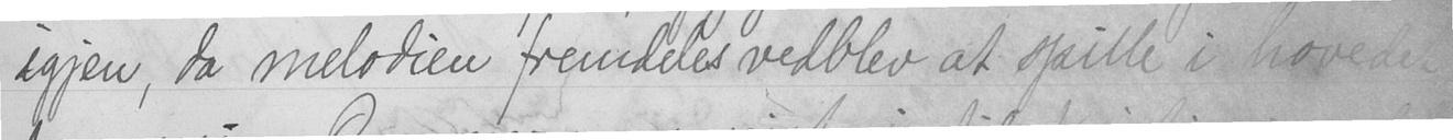igjen, da melodien fremdeles vedblev at spille i hovedet
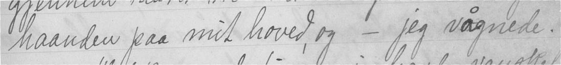haanden paa mit hoved, og - jeg vågnede.
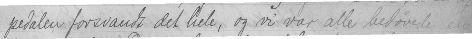pedalen forsvandt det hele, og vi var alle bedøvede en
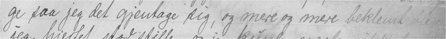ge saa jeg det gjentage sig, og mere og mere beklemt blev
